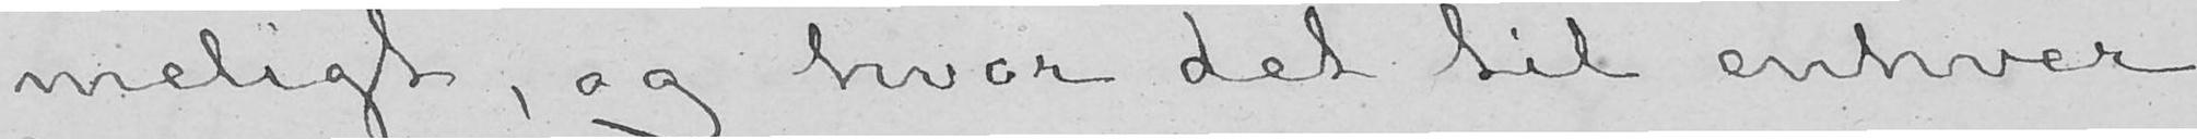meligt, og hvor det til enhver
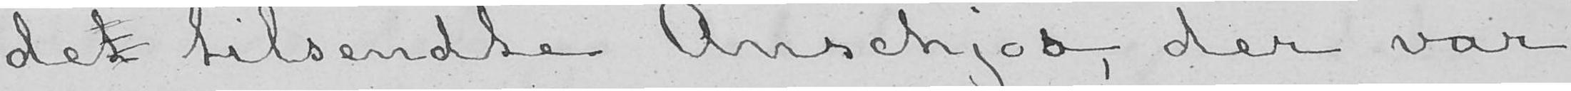det tilsendte Anschjos, der var
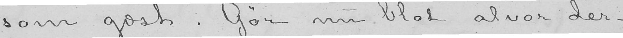som gæst. Gør nu blot alvor der-
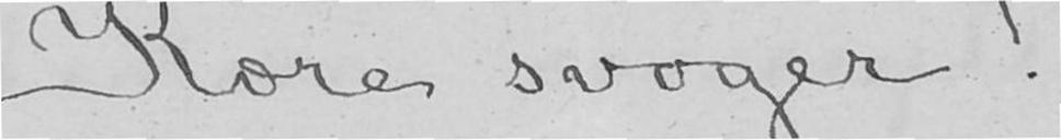Kære svoger!
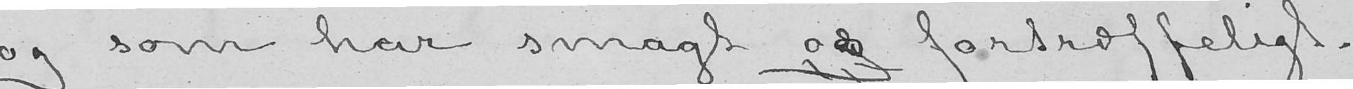og som har smagt ogs fortræffeligt.
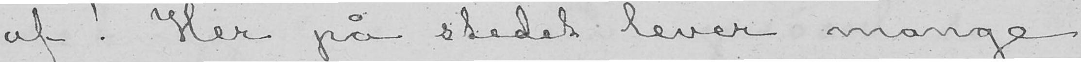af! Her på stedet lever mange
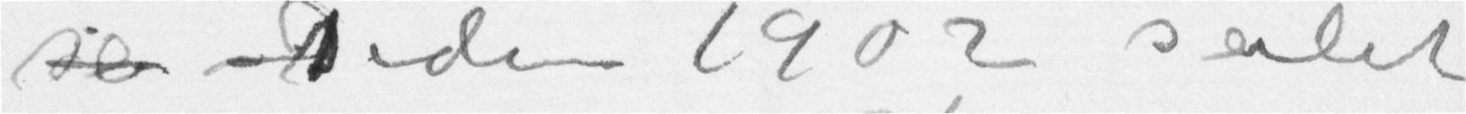se siden 1902 seilet
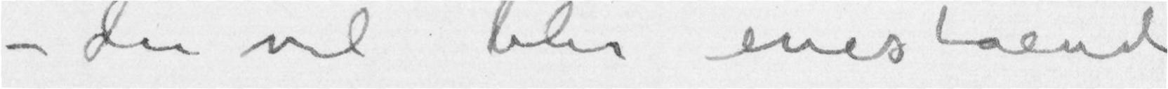– der vel blir enestående
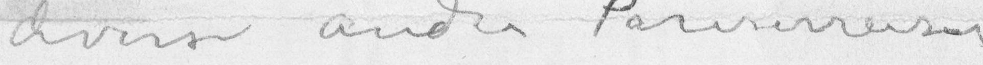diverse andre Pariserreiser
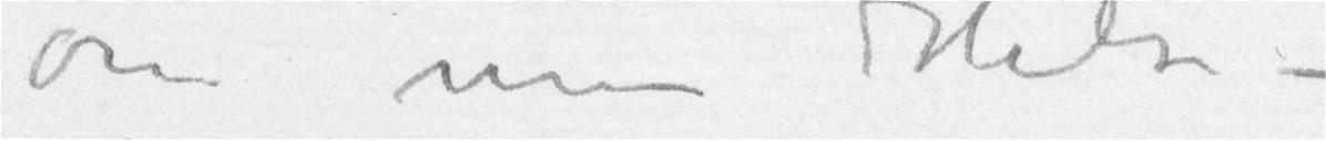om min Helse –
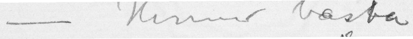– Hermed basta
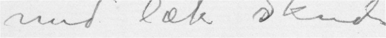med læk Skude
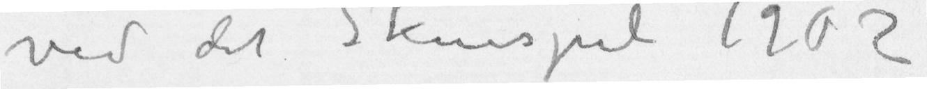ved det Skuespil 1902
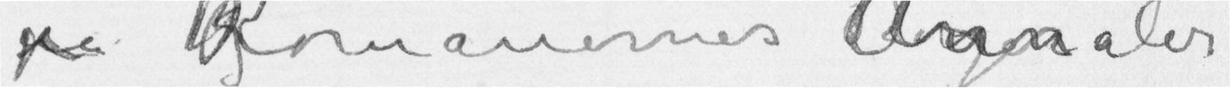påi Romanernes Annaler
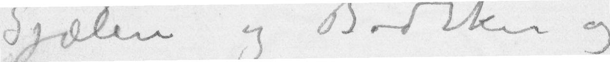Sjælen og Bødtker og
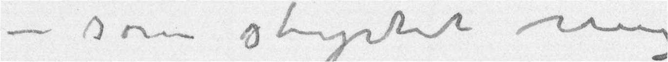– som styrtet mig
